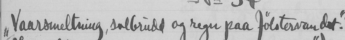"Vaarsmeltning, solbrudd og regn paa Jølstervandet".
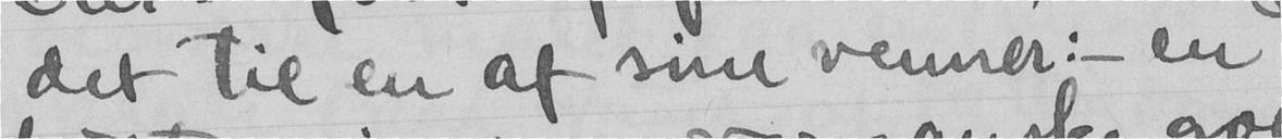det til en af sine venner : - en
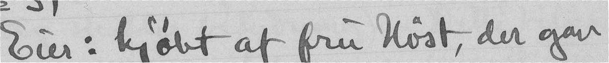Eier: kjøbt af fru Høst, der gav
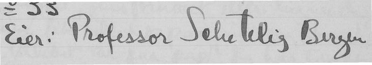Eier: Professor Schetelig Bergen
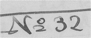No 32
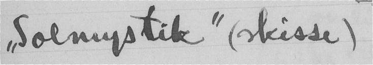"Solmystik" (skisse)
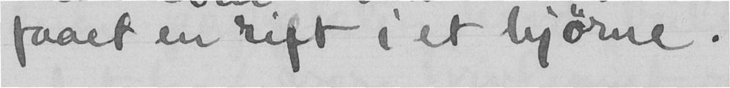faaet en rift i et hjørne.
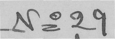No 29
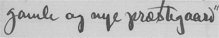gamle og nye præstegaard"
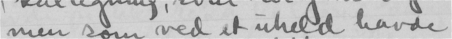men som ved et uheld havde
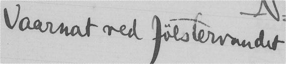Vaarnat ved Jølstervandet
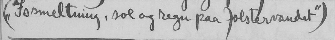("Issmeltning i sol og regn paa Jølstervandet")
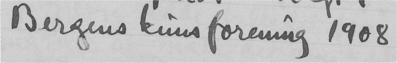Bergens kunsforening 1908
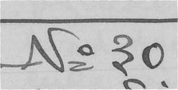No 30
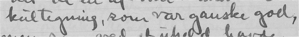kultegning, som var ganske god,
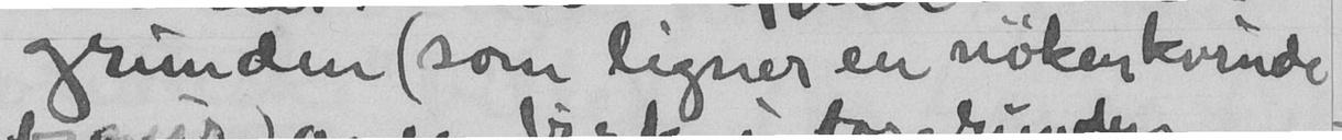grunden (som ligner en nøken kvinde
